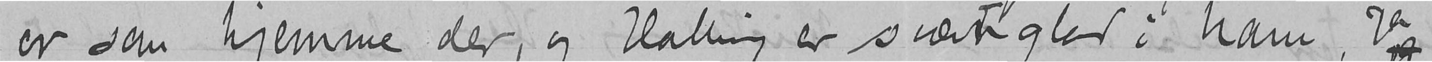er som hjemme der, og Halling er svært glad i ham, jeg
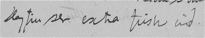Dagfin ser extra frisk ud.
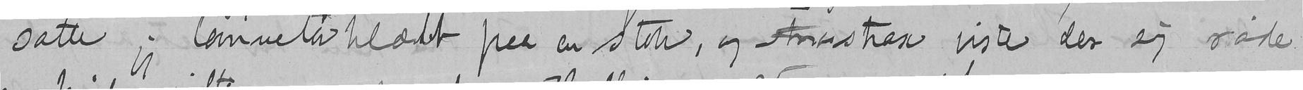satte jeg lommetørklædet paa en stok, og strrstrax viste der sig røde
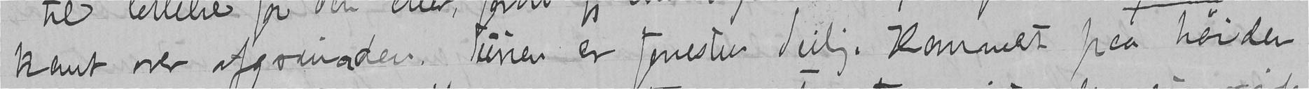kant over afgrunden. Turen er forresten deilig. Kommet paa høiden
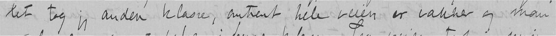let tog jeg anden klasse, omtrent hele veien er vakker og man
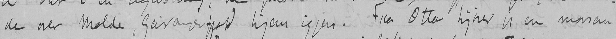de over Molde, Geirangerfjord hjem igjen. Fra Otta kjører jeg en morsom
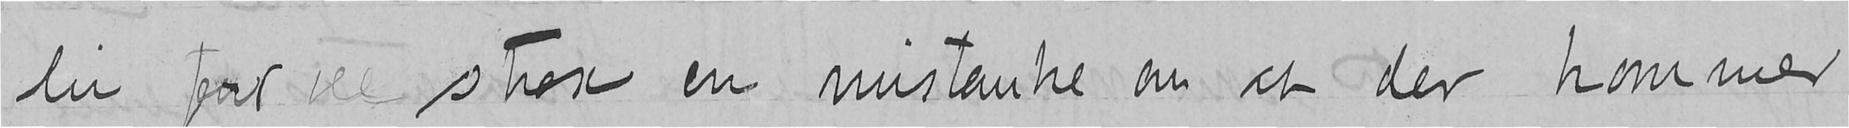Du faar vel strax en mistanke om at der kommer
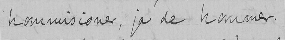kommisioner, ja de kommer.
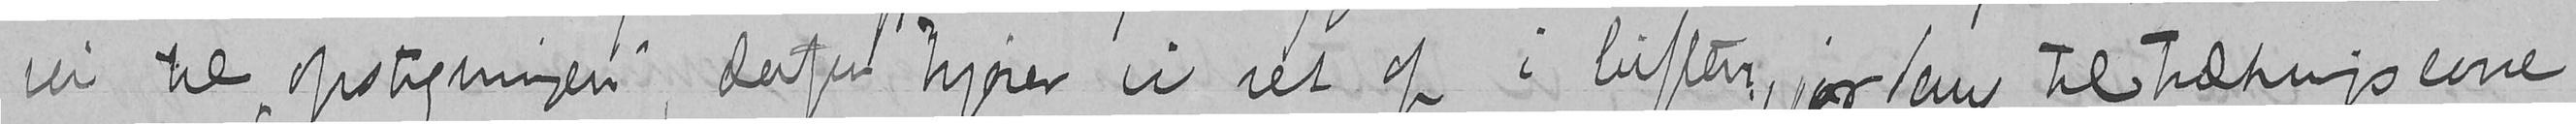vei til "opstigningen", derfra kjører vi ret op i luften, for dens tiltrækningsevne
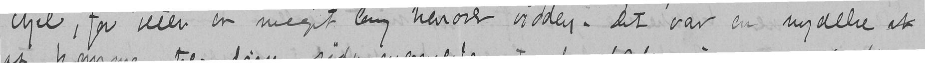ihjel, for veien er meget lang henover vidden. Det var en nydelse at
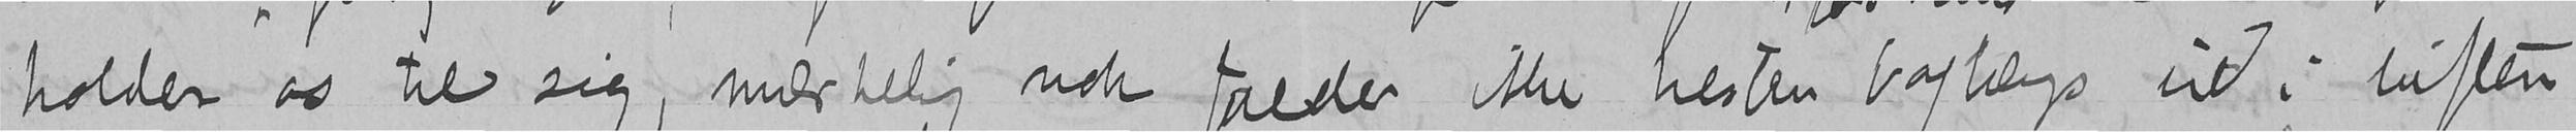holder os til sig, mærkelig nok falder ikke hesten baglængs ud i luften
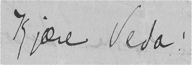Kjære Veda,
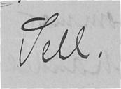Tell.
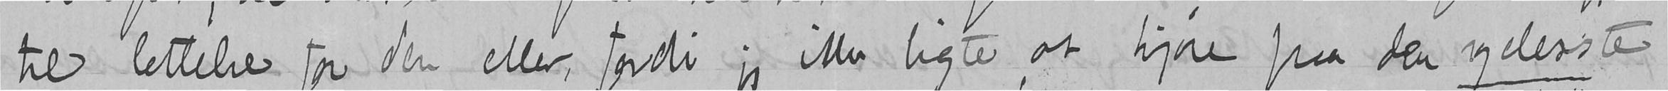til lettelse for den eller, fordi jeg ikke ligte at kjøre paa den yderste
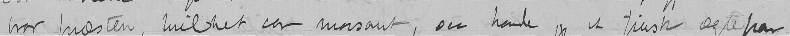bror præsten, hvilket var morsomt, saa havde jeg et finsk Ægtepar
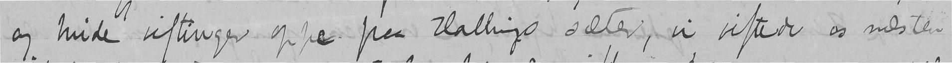og hvide viftinger oppe paa Hallings sæter, vi viftede os næsten
